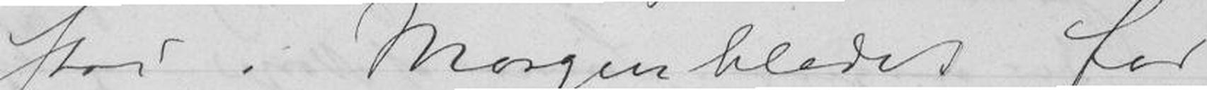stod i Morgenbladet for
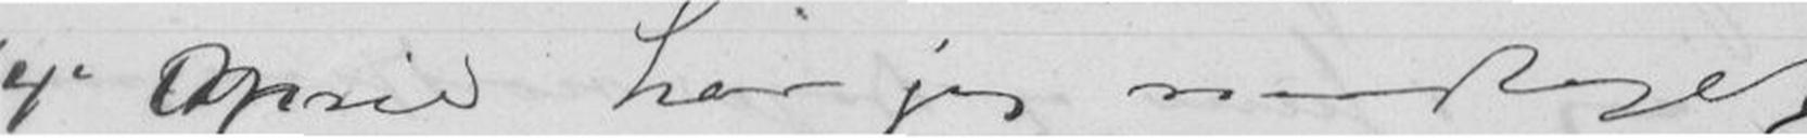14 April har jeg modtaget
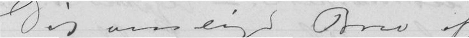Dit venlige Brev af
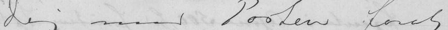dig med Posten forat
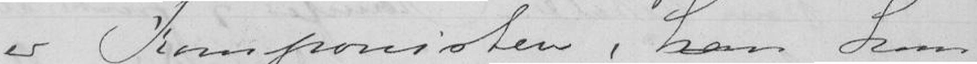er Komponisten, han kom
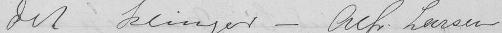det klinger - Alfr. Larsen
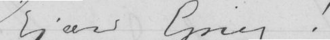Kjære Grieg!
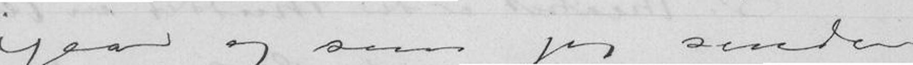igaar og som jeg sender
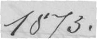1873.
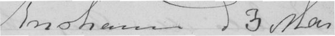Kristiania d 3 Mai
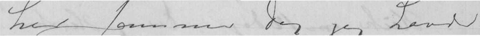her samme dag jeg havde
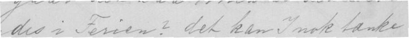des i Ferien? det kan I nok tænke
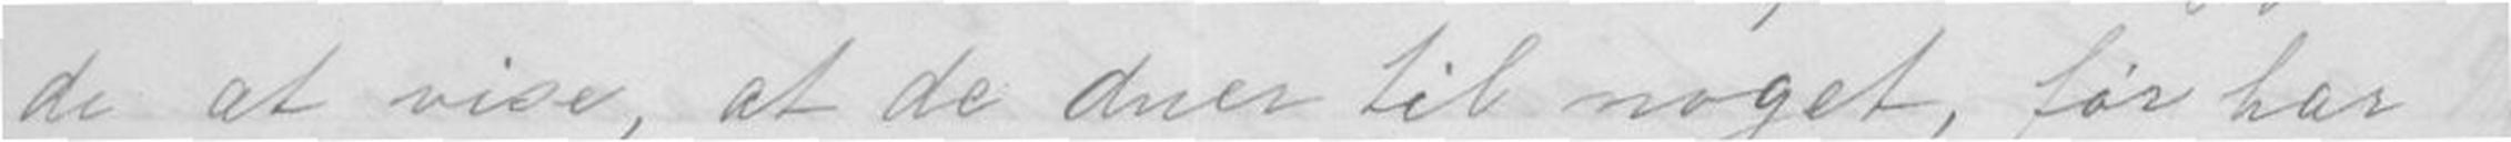de at vise, at de duer til noget, før har
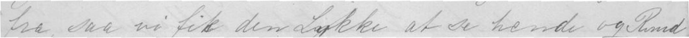fra, saa vi fik den Lykke at se hende og Prud
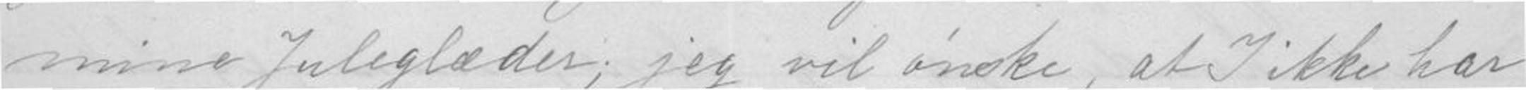mine Juleglæder; jeg vil ønske , at I ikke har
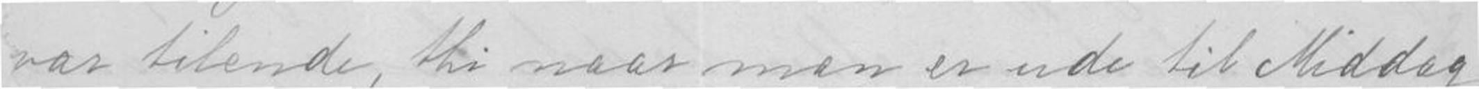var tilende, thi naar men er ude til Middag
